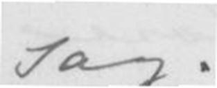sag.
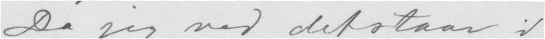Da jeg ved det staar i
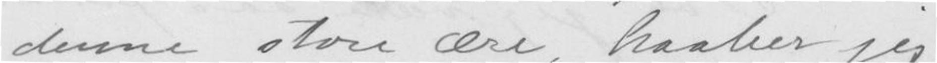denne store ære, haaber jeg
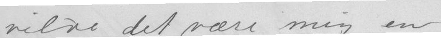vilde det være mig en
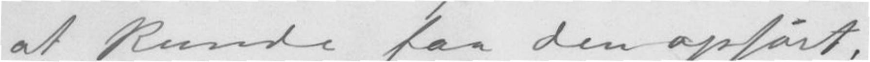at kunde faa den opført,
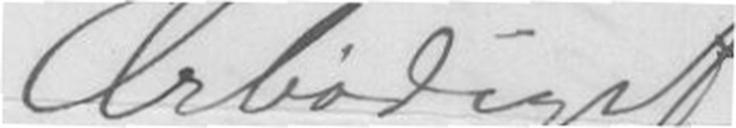Ærbødigst
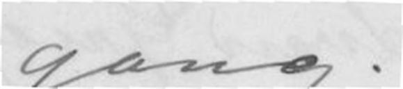gang.
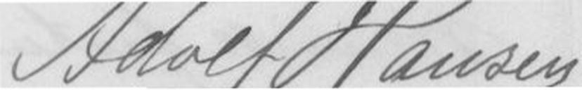Adolf Hansen
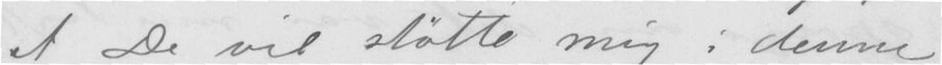at De vil støtte mig i denne
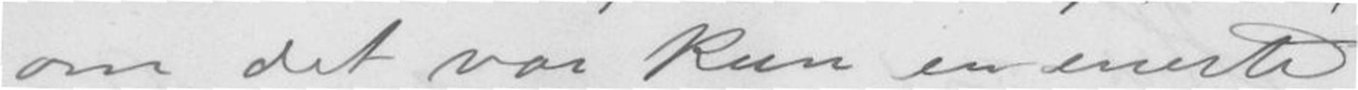om det var kun en eneste
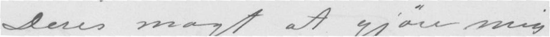Deres magt at gjøre mig
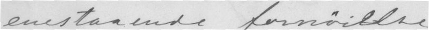enestaaende fornøielse
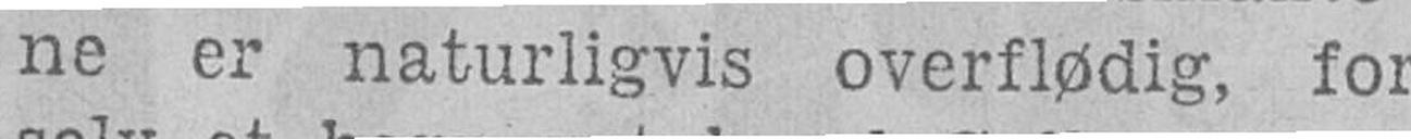ne er naturligvis overflødig, for
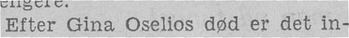Efter Gina Oselios død er det in-
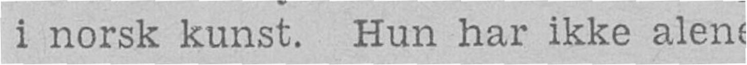i norsk kunst. Hun har ikke alene
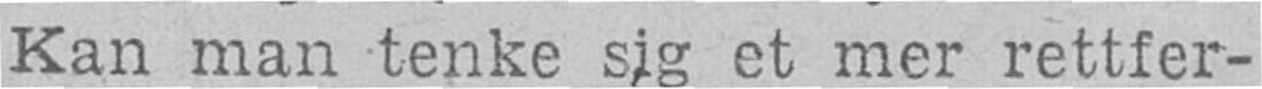Kan man tenke sig et mer rettfer-
